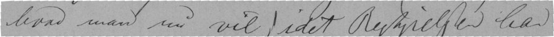hvad man nu vil) idet Beskjellsken har
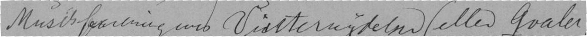Musikforeningens Vissternydelser. (eller qvaler,
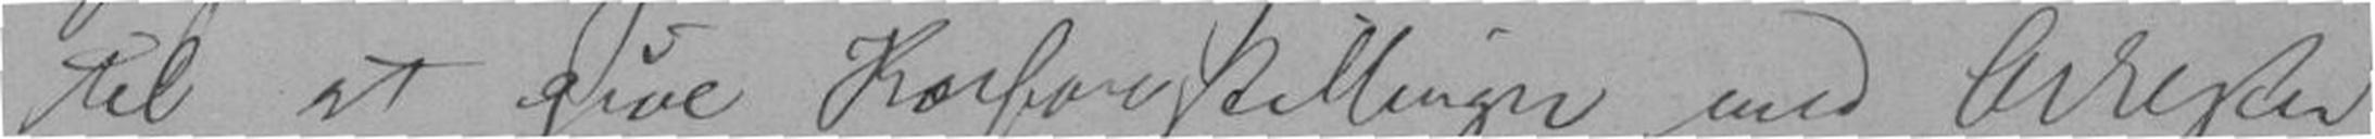til at give Korforestillinger med Orkester
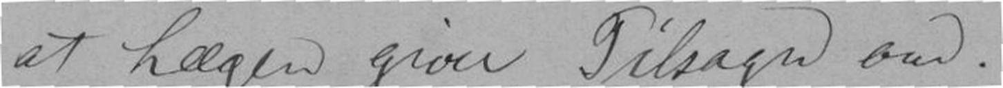at Lægen giver Tilsagn om.
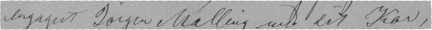engagert Jørgen Malling med sit Kor,
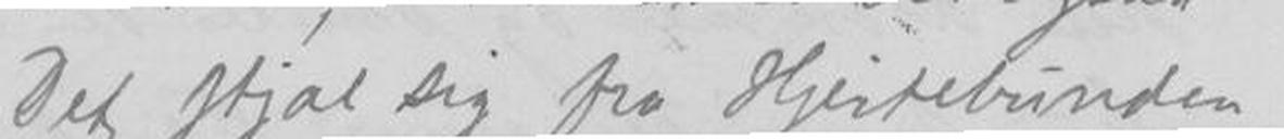Det stjal sig fra Hjertebunden.
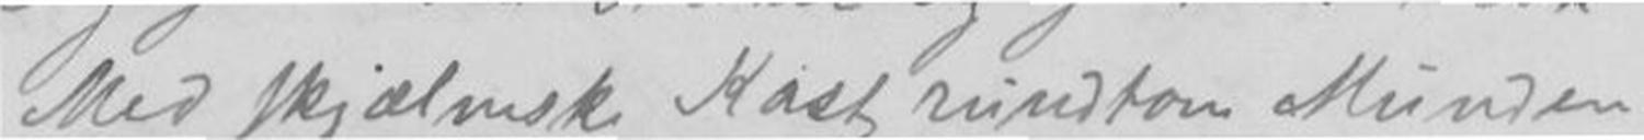Med skjælmsk Kast rundtom Munden
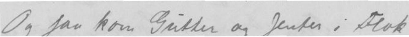Og saa kom Gutter og Jenter i Flok
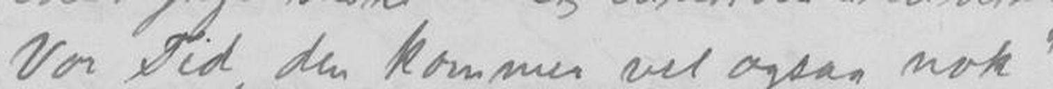Vor Tid, den kommer vel ogsaa nok -
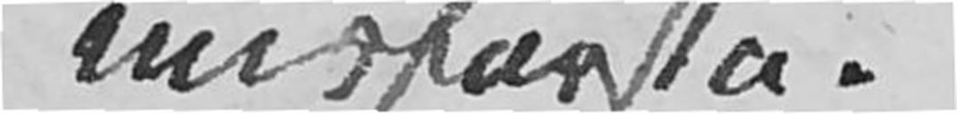misforstå.
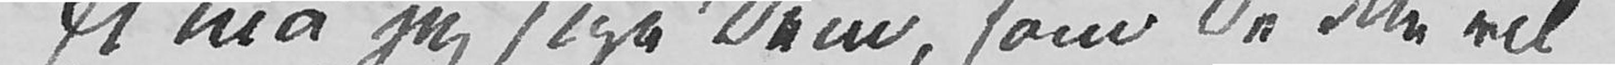Et må jeg sige Dem, som De ikke vil
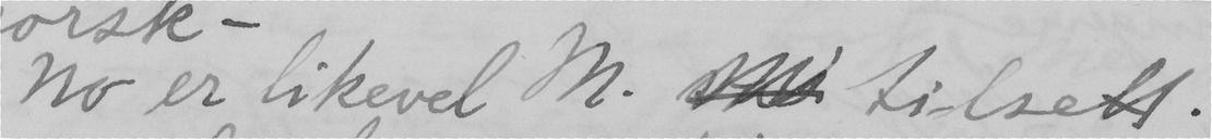No er likevel M. tilsett.
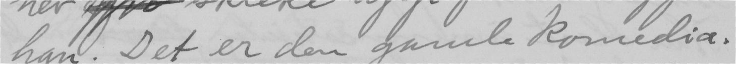han. Det er den gamle komedia.
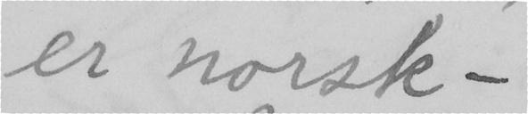er norsk.
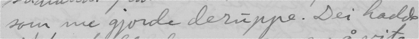som me gjorde deruppe. Dei hadde
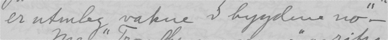er utruleg vakne i bygdene no".
